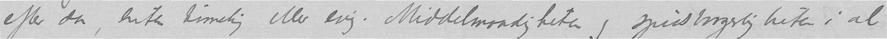efter da, enten timelig eller evig. Middelmaadighetenr og spissborgerligheten i al
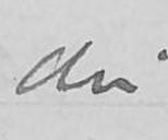din
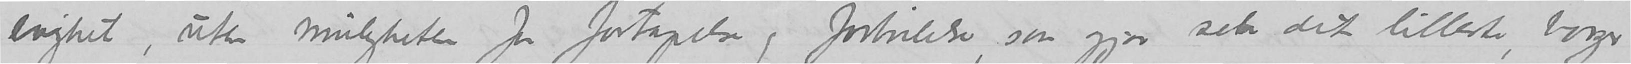evighet, uten muligheten for fortapelse og fortvilelse, som gjør selv det lilleste, borg-
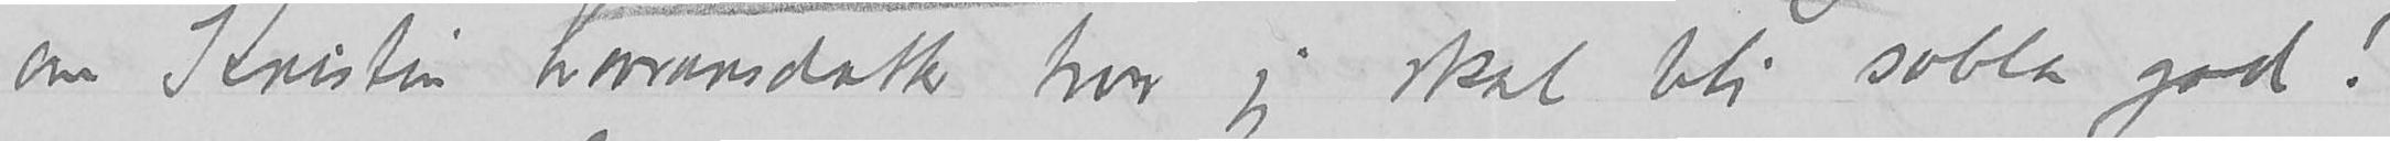om Kristin Lavransdatter tror jeg skal bli sabla god!
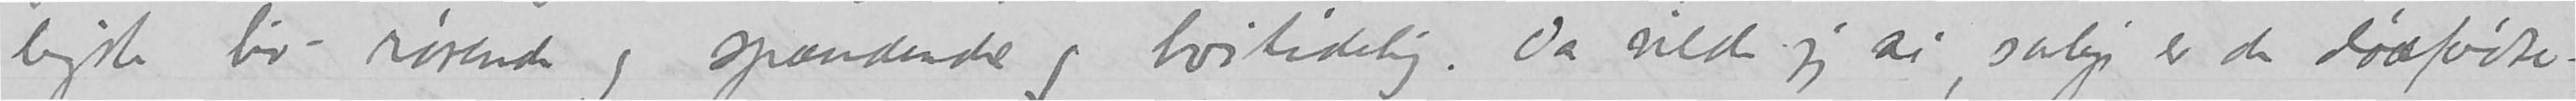erligste liv, rørende og spændende og høitidelig. Da vilde jeg si, salige er de dødfødte -
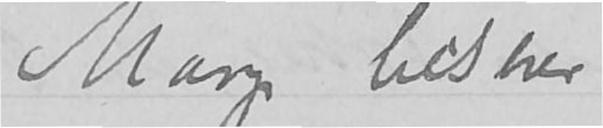Mange hilsener
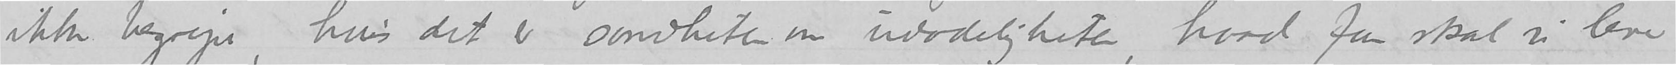ikke begripe, hvis det er sandheten om udødeligheten, hvad for skal vi leve
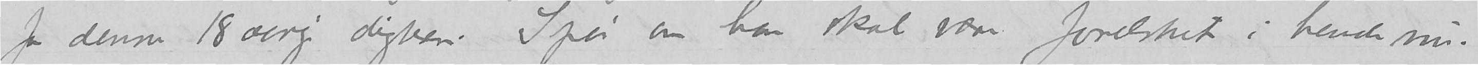for denne 18 årige digteren. Spør om han skal være forelsket i hende nu.
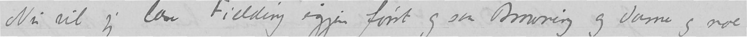Nu vil jeg læse Fielding igjen først og sa Browning og Donne og noe
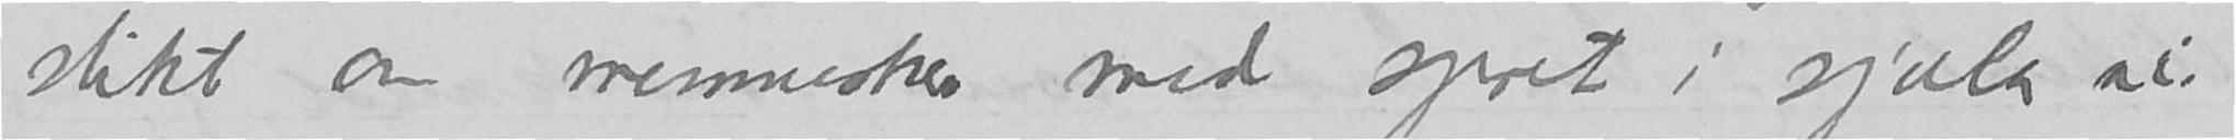slikt om mennesker med spret i sjæla si.
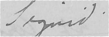Sigrid
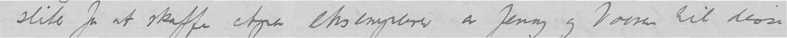Jeg sliter for at skaffe etpar eksemplarer av Jenny og Vaaren til disse
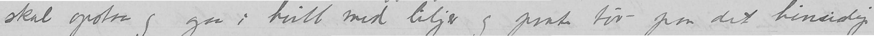skal opstaa og gaa i hvitt med liljer og pent tøi paa det hinsidige
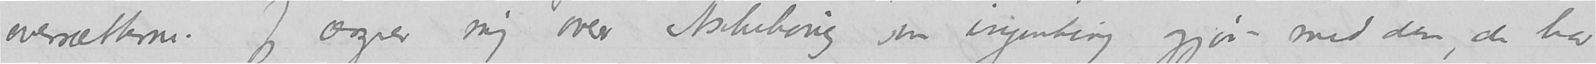oversætterne. Jeg ærgrer mig over Aschehoug som ingenting gjør med dem, de har
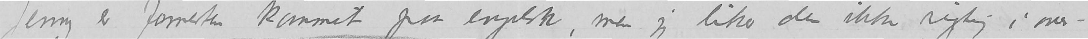Jenny er forresten kommet paa engelsk, men jeg liker den ikke rigtig i over-
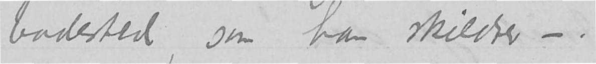badested, som han skildrer -.
